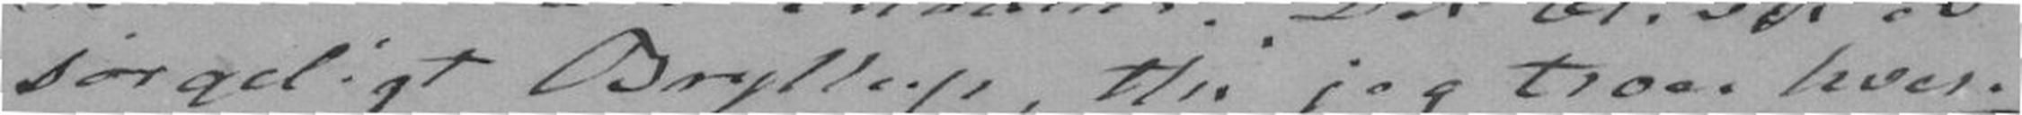sørgeligt Bryllup, thi jeg troer hver-
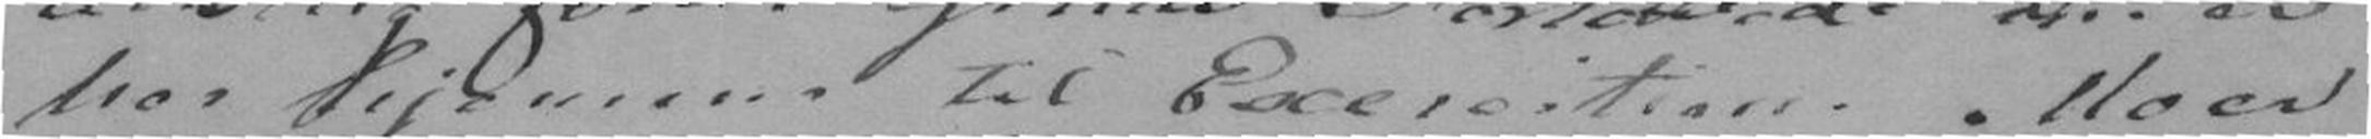her hjemme til Exercition. Moer
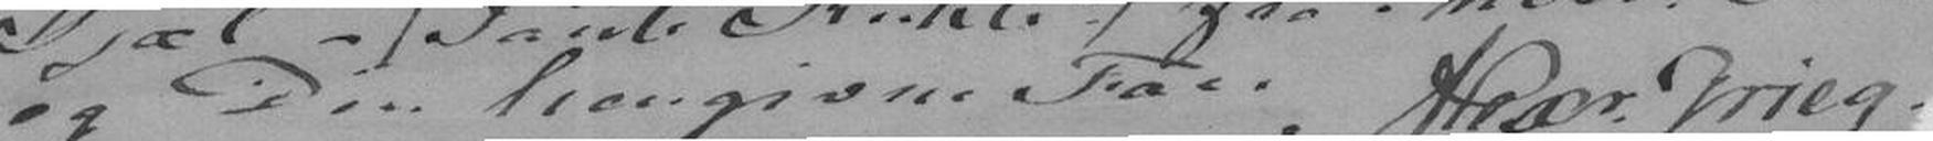og Din hengivne Faer Alexr. Grieg.
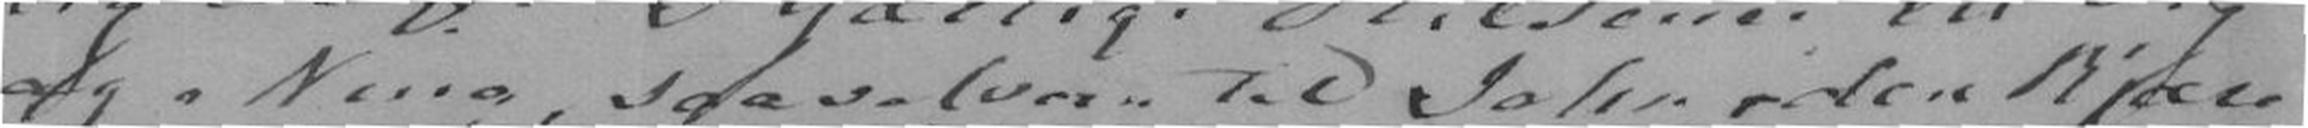og Nina, saavelsom til John den kjære
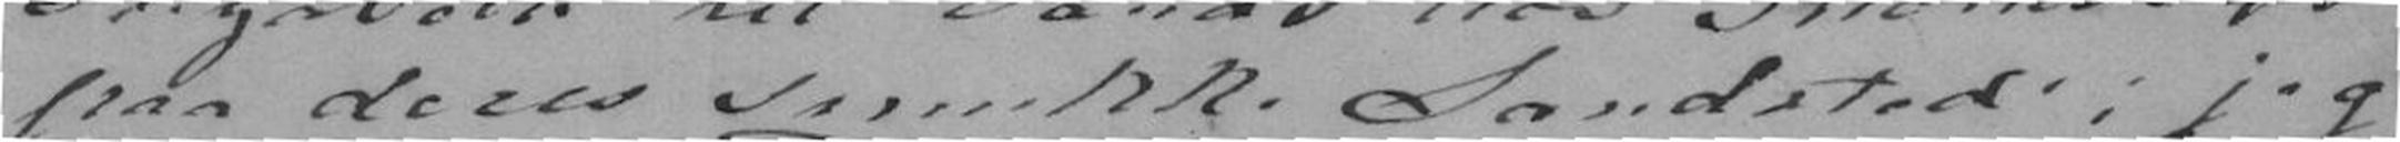paa deres smukke Landsted: jeg
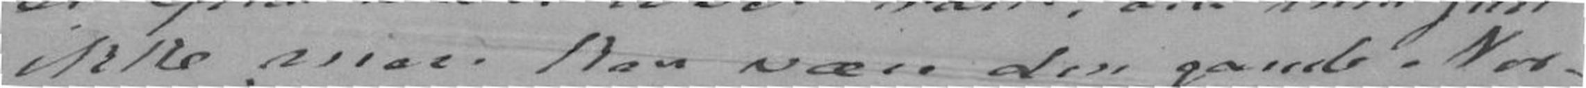ikke mere kan være den gamle Nor-
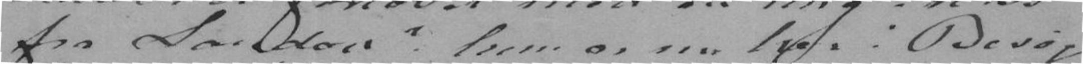fra London? hun er nu her paa Besøg.
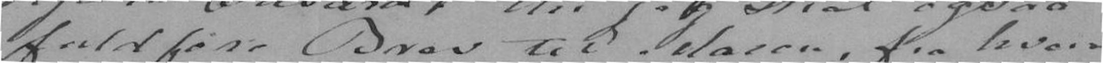fuldføre Brev til Maren, fra hvem
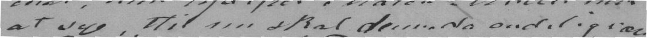at sye, thi nu skal denne da endelig være
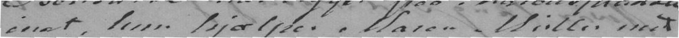inat, hun hjælper Maren Müller med
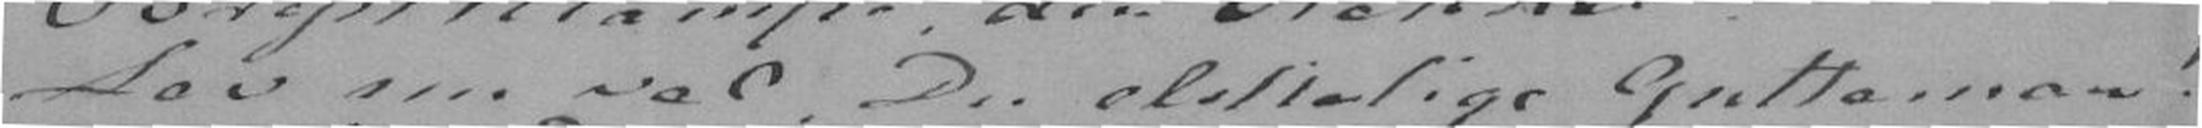Lev nu vel Du elskelige Gutteman!
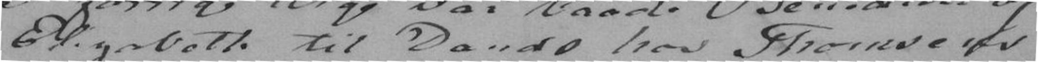Elizabeth til Dands hos Thomsens
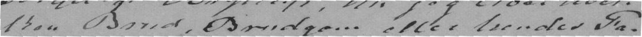ken Brud, Brudgom eller hendes Fa-
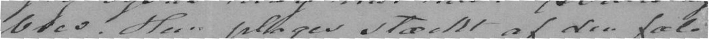brev. Hun plages stærk af den fæle
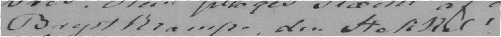Brystkrampe, den Stakkels!
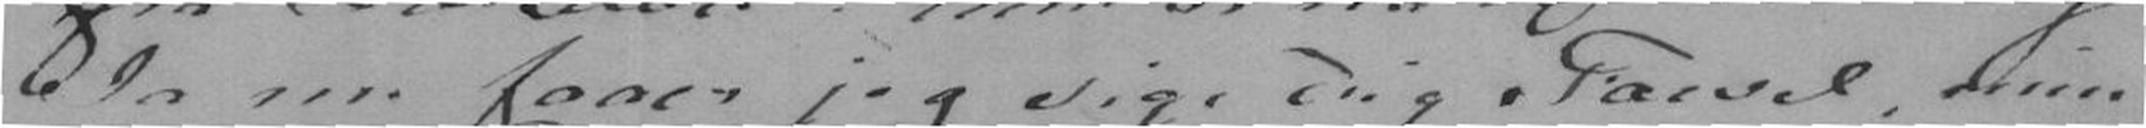Ja nu faaer jeg sige Dig Farvel, min
